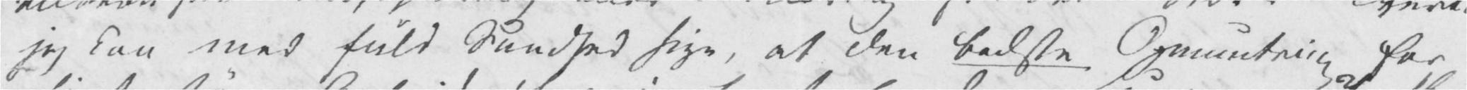jeg kan med fuld Sandhed sige, at den bedste Opmuntring for
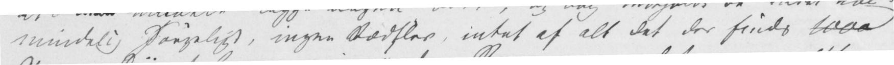kunde
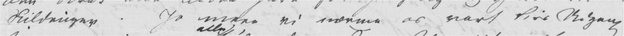har følgelig stridt og lidt sit hele Liv igjennem og skjøndt nu hvert Pulsslag i hende vidner at hvad der er skildret i Fortællingen er sandt, sande og for Enhver der ikke blot
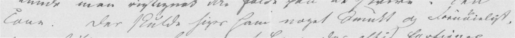Tone. Der skulde siges ham noget Smukt og Fornøieligt,
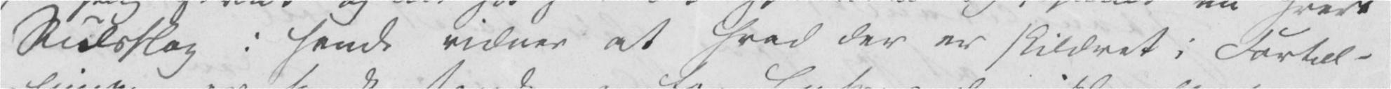meget forbundne
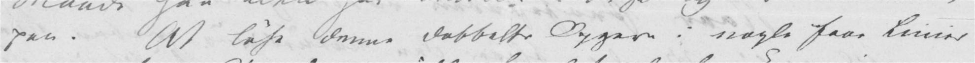paa. At løse denne dobbelte Opgave i nogle faae Linier
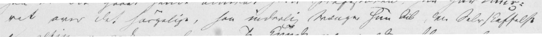er kun et
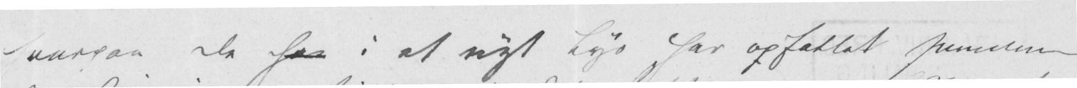hvorpaa De har i et nyt Lys har opfattet
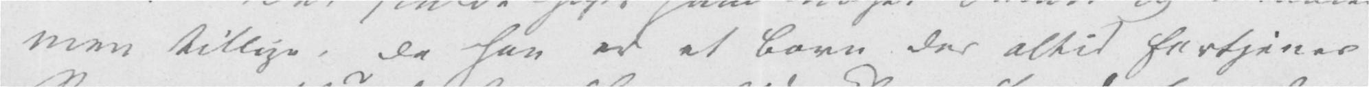men tillige, da han er et Barn der altid fortjener
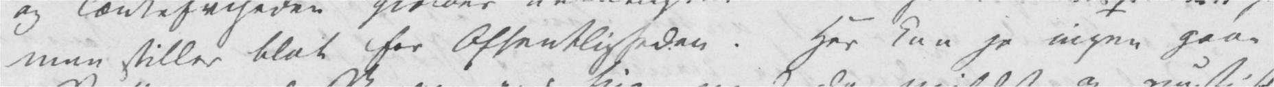man stiller blot for Offentligheden. Her kan jo ingen gaae
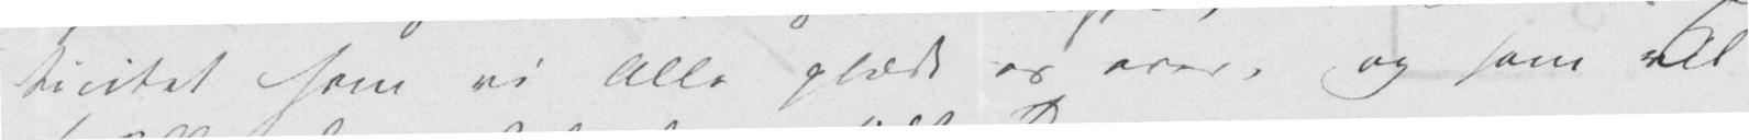som vi Alle
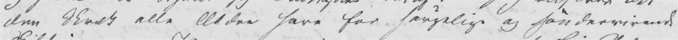Dame passer ikke paa hende da hun aldrig kan blive gammel – hun har mange af de Egenskaber som Professoren udhæver som eiendommelige for en Digtersjel, hun
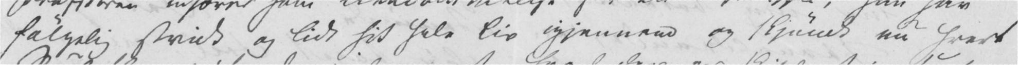forsikret mig om at De har læst det med Velvillie, og at Freden er ganske oprettet mellem Dem og Deres
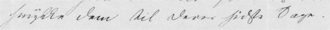smykke Dem til Deres sidste Dage.
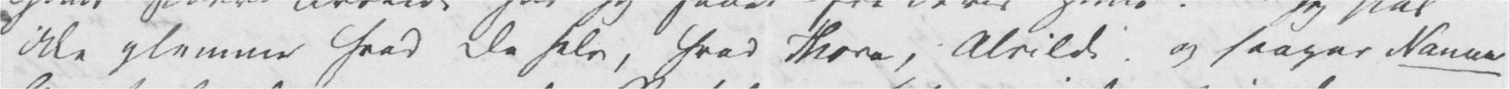ikke glemme hvad De selv, hvad Thora, Alvilde og saagar Nanna
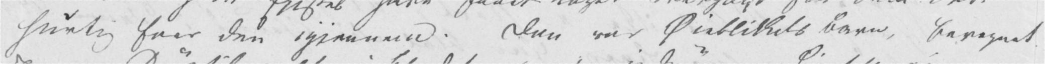hurtig foer den igjennem. Den var Øieblikkets Barn, beregnet
